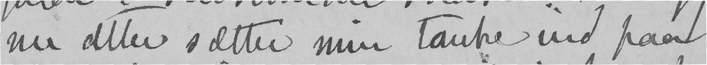nu atter sætter min tanke ind paa
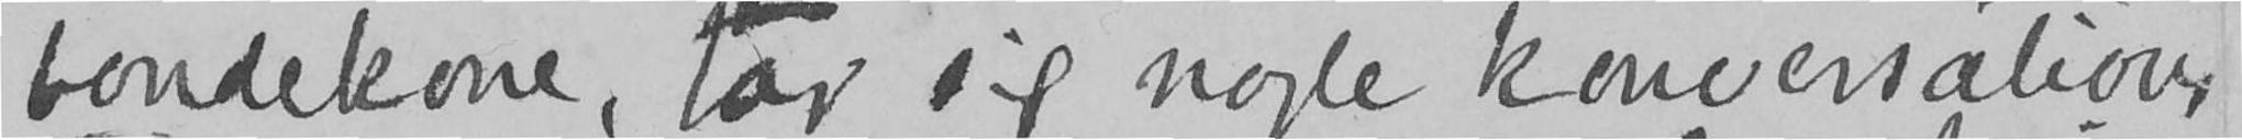bondekone, tar sig nogle konversations-
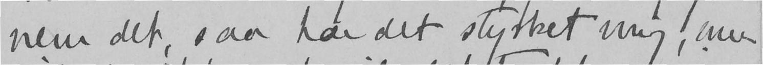nem det, saa har det styrket mig, men
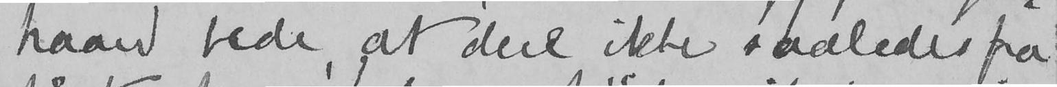haand bede, at dere ikke saaledes fra
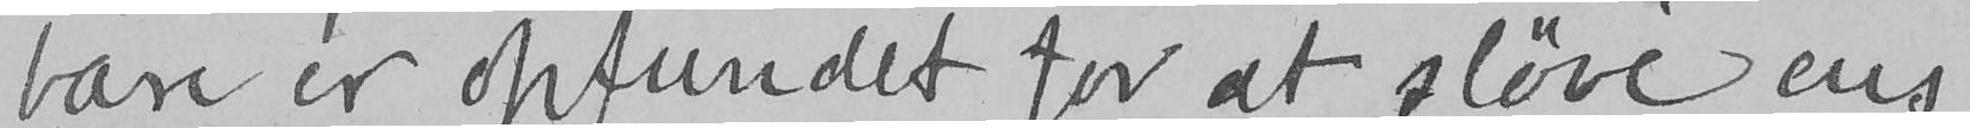bare er opfundet for at sløve ens
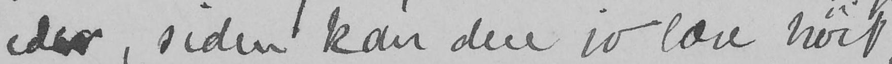eder, siden kan dere jo læse høit.
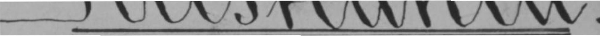Kristiania.
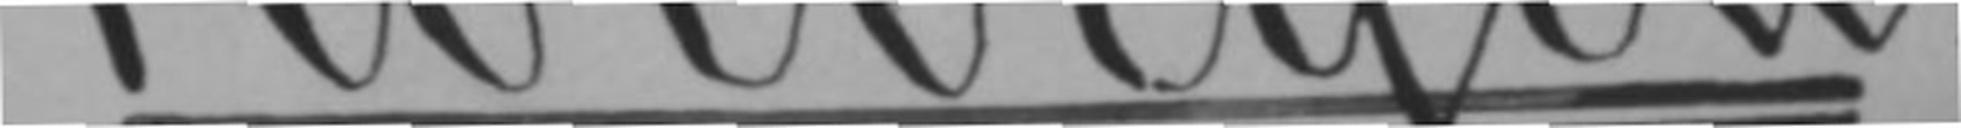(Norvegia.)
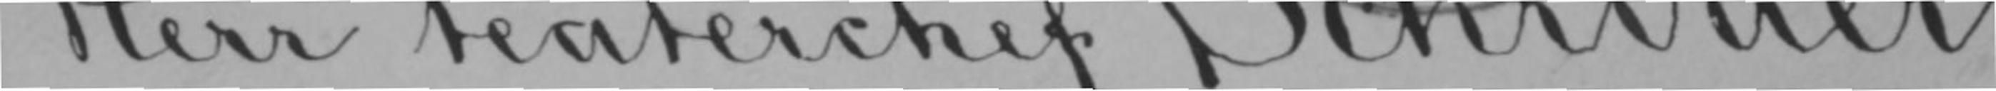Herr teaterchef Schrøder,
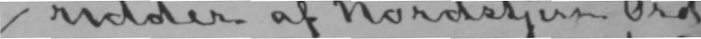ridder af Nordstjerne Ord
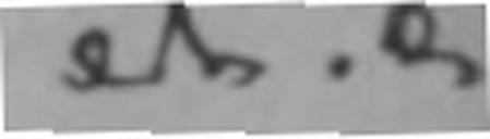et. c
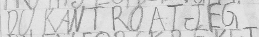DU KAN TRO A JEG
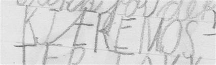KJÆRE MOS-
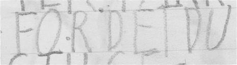FOR DET DU
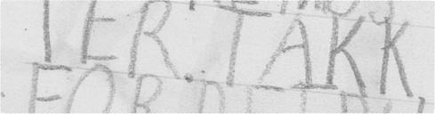TER. TAKK
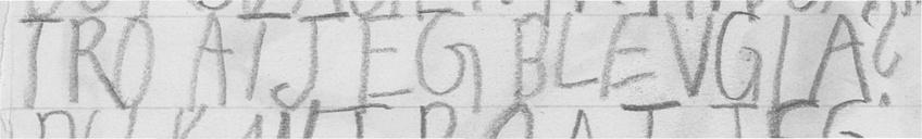TRO AT JEG BLEV GLA?
